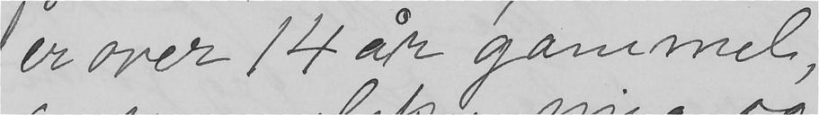er over 14 år gammel,
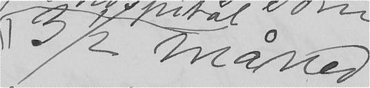3 1/2 måned
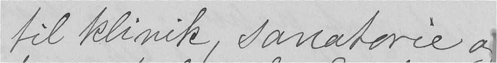til klinik, sanatorie og
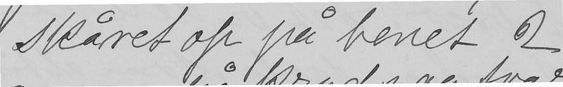skåret op på benet 2
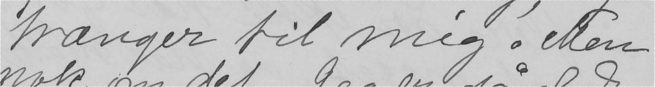trænger til mig. Men
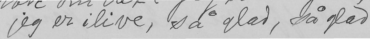jeg er ilive, så glad, så glad
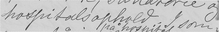hospitalsophold. I som-
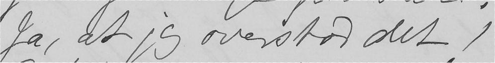Ja, at jeg overstod det!
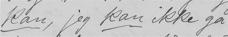kan, jeg kan ikke gå
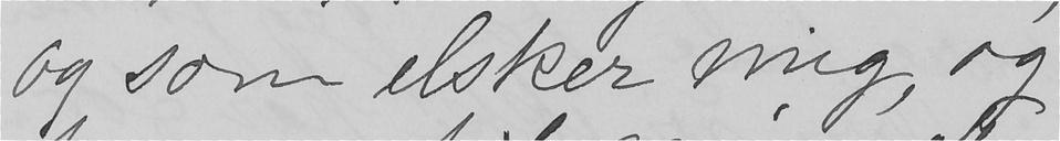og som elsker mig, og
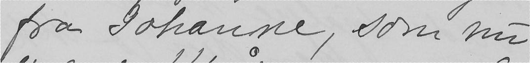fra Johanne, som nu
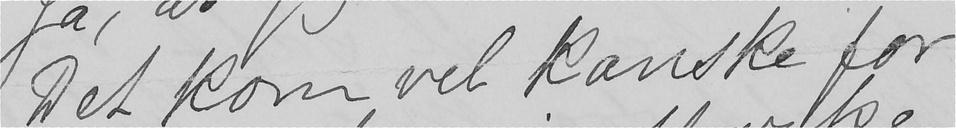Det kom vel kanske for
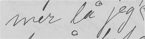mer lå jeg
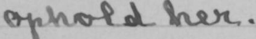ophold her.
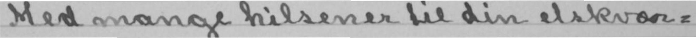Med mange hilsener til din elskvær-
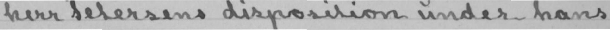herr Petersens disposition under hans
